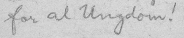for al Ungdom!
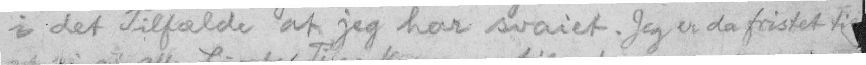i det Tilfælde at jeg har svaiet. Jeg er da fristet til
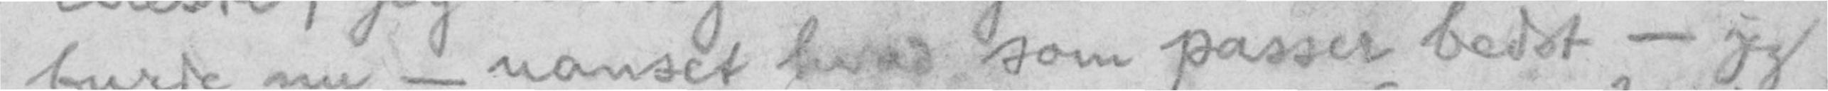burde nu - uanset hvad som passer bedst - jeg
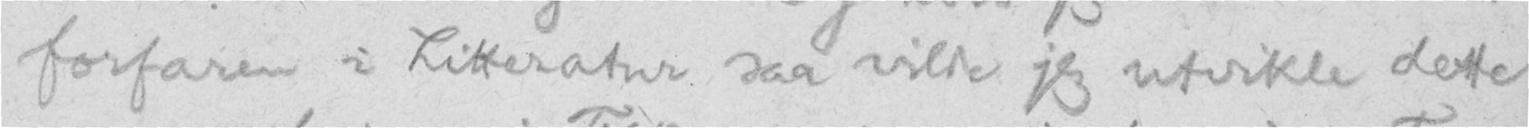forfaren i Litteratur saa vilde jeg utvikle dette
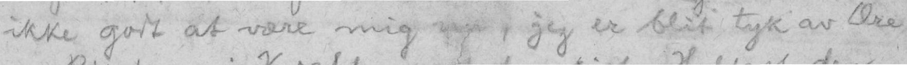ikke godt at være mig nu, jeg er blit tyk av Ære
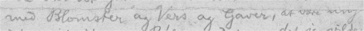med Blomster og Vers og Gaver, at være ung
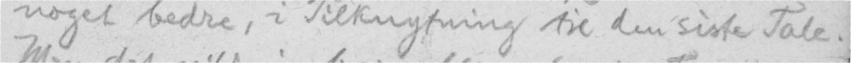noget bedre, i Tilknytning til den siste Tale.
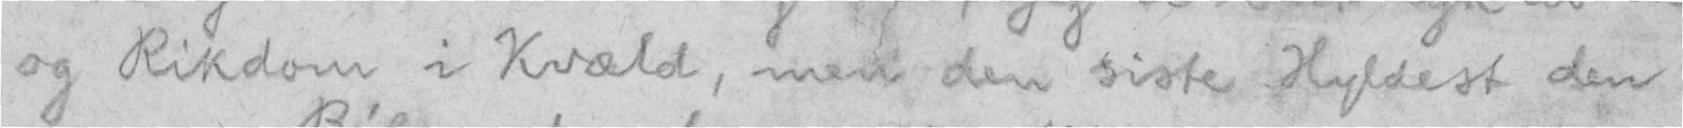og Rikdom i Kvæld, men den siste Hyldest den
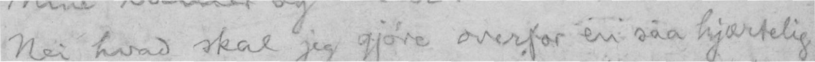Nei hvad skal jeg gjøre overfor en saa hjærtelig
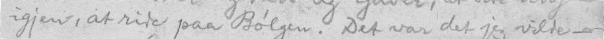igjen, at ride paa Bølgen. Det var det jeg vilde -
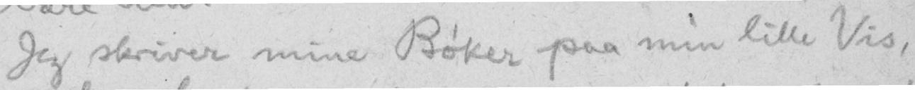Jeg skriver mine Bøker paa min lille Vis,
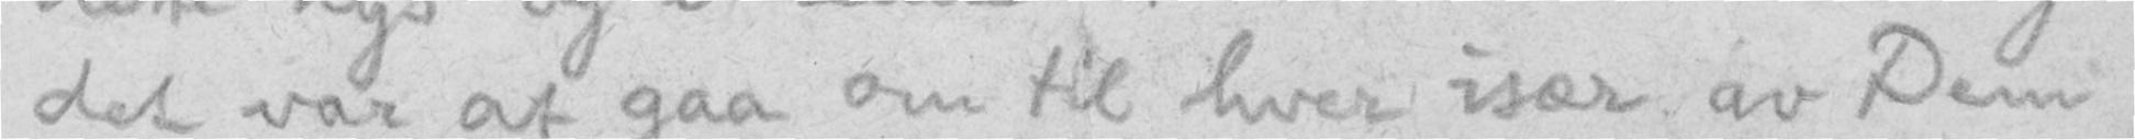det var at gaa om til hver især av Dem
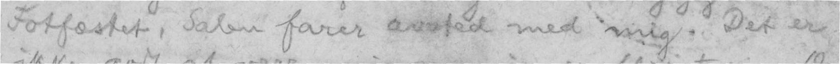Fotfæstet, Salen farer avsted med mig. Det er
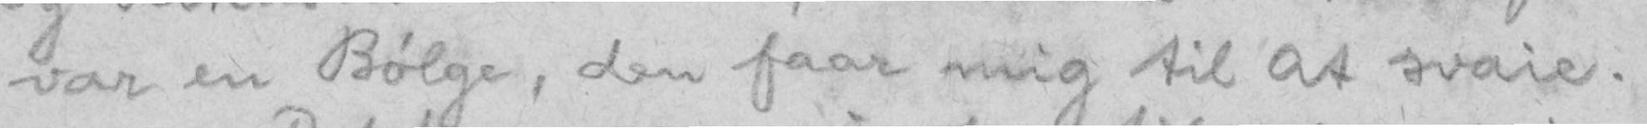var en Bølge, den faar mig til at svaie.
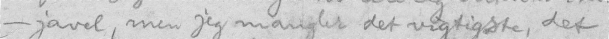- javel, men jeg mangler det vigtigste, det
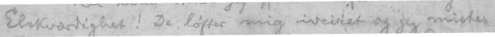Elskværdighet! De løfter mig iveiret og jeg mister
